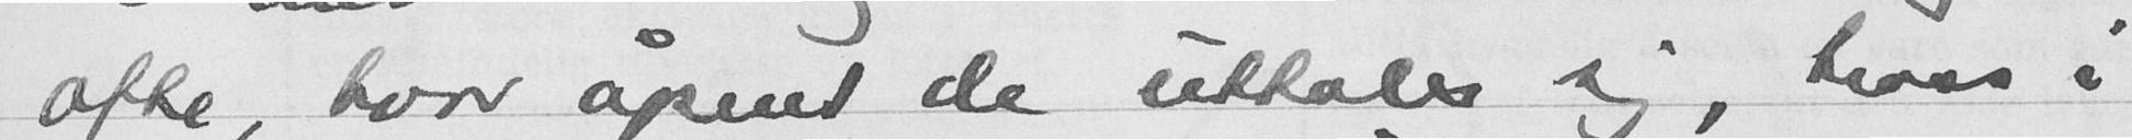ofte, hvor åpent de uttaler sig, tross i
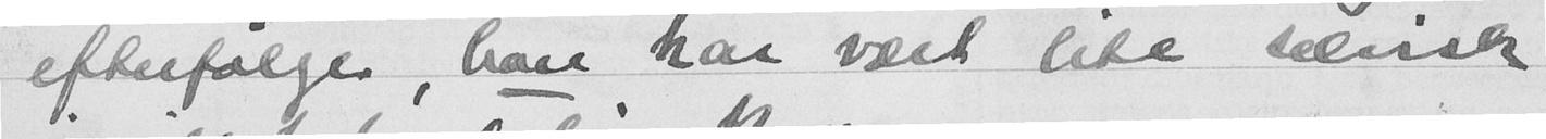efterfølger, han har vært lite selvisk
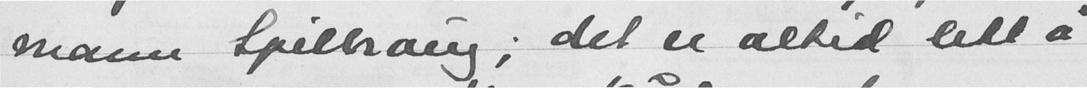mann Spilhaug; det er altid lett å
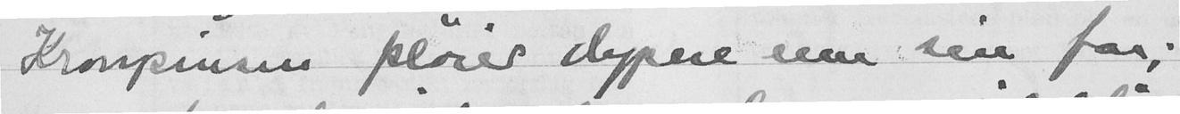Kronprinsen pløier dypere enn sin far;
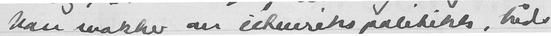han snakker om utenrikspolitikk, både
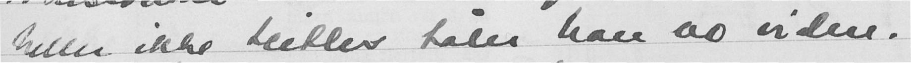heller ikke Hitler tåler han no videre.
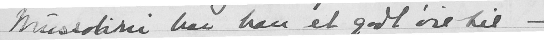Mussolini har han et godt øie til -
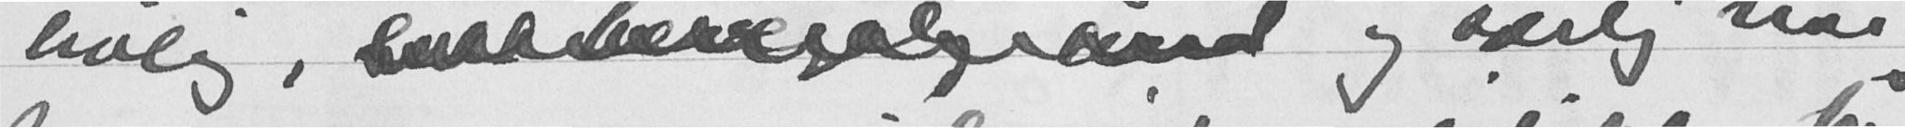livlig,  og særlig når
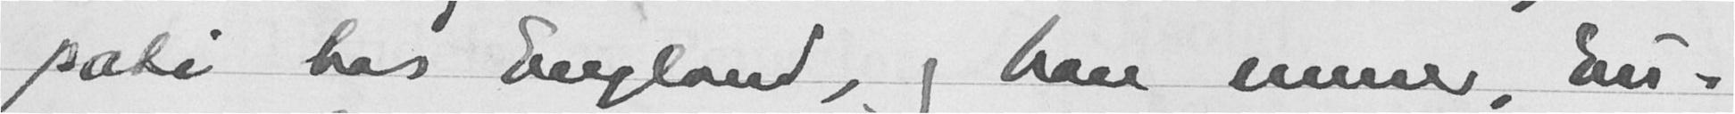pati hos England, og han mener, Eu-
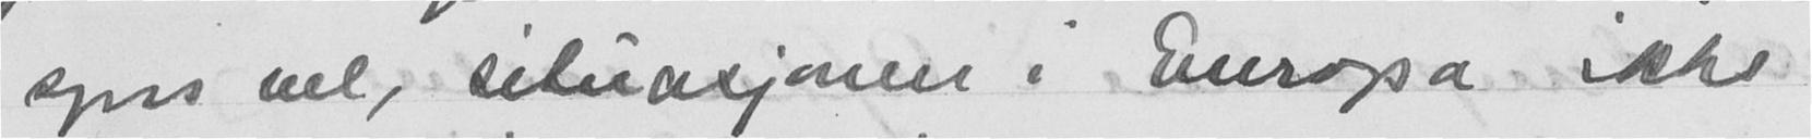syns vel, situasjonen i Europa ikke
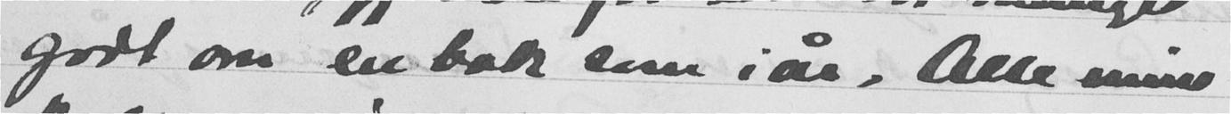godt om en bok som iår. Alle mine
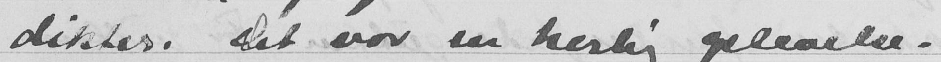dikter. Det var en herlig oplevelse.
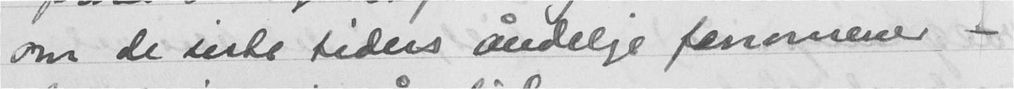om de siste tiders åndelige fenomener -
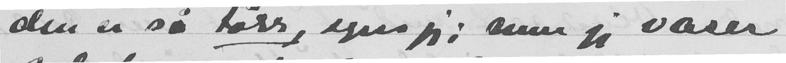den er så tørr, synes jeg; men jeg vaser
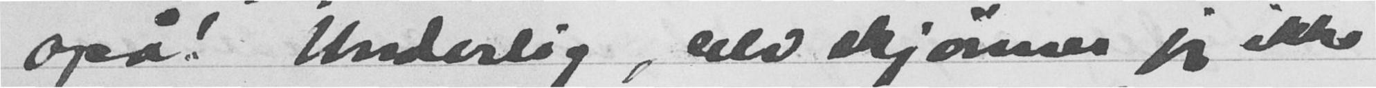også! Underlig, selv skjønner jeg ikke
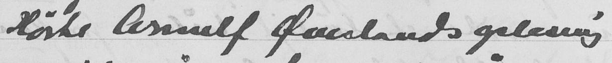Hørte Arnulf Øverlands oplesing
